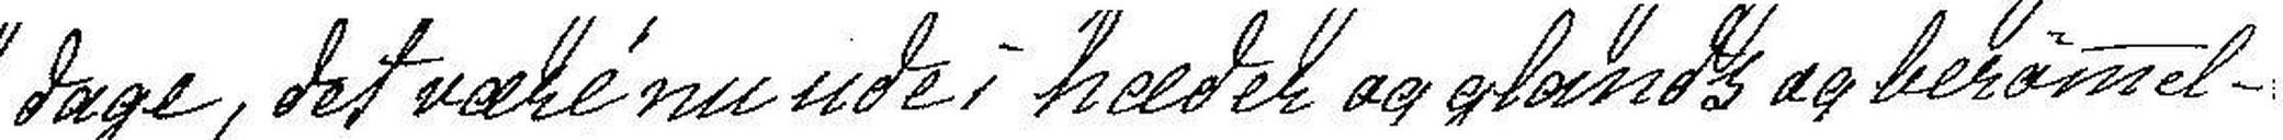dage, det være nu ude i hæder og glands og berømel-
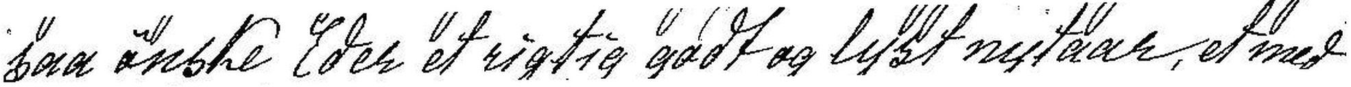saa ønske Eder et rigtig godt og lyst nytaar, et med
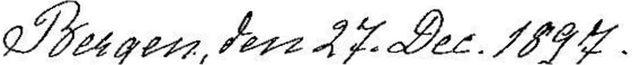Bergen, den 27. Dec. 1897.
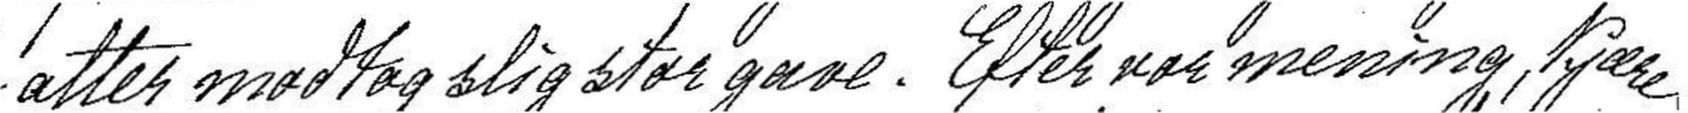atter modtog slig stor gave. Efter vor mening, kjære
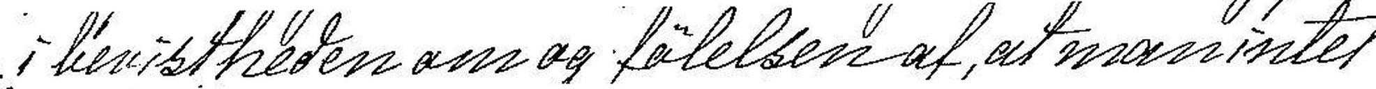i bevistheden om og følelsen af, at man intet
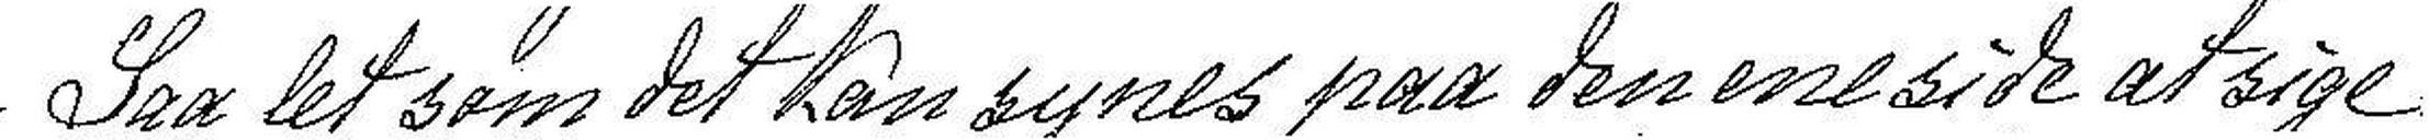Saa let som det kan synes paa den ene side at sige
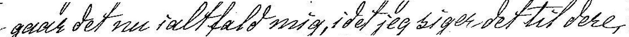gaar det nu ialtfald mig, idet jeg siger det til dere,
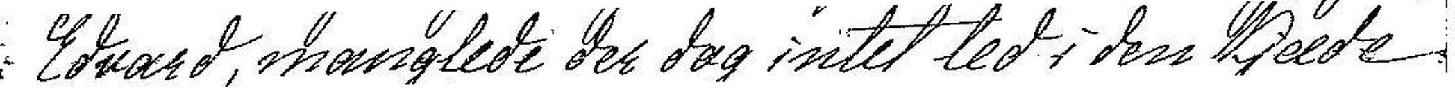Edvard, manglede der dog intet led i den Kjæde
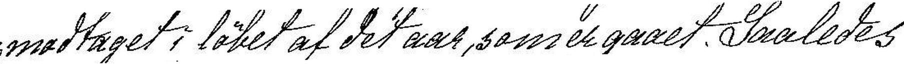modtaget i løbet af det aar, som er gaaet. Saaledes
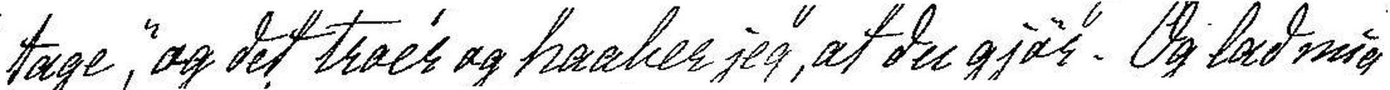tage, og det troer og haaber jeg, at du gjør. Og lad mig
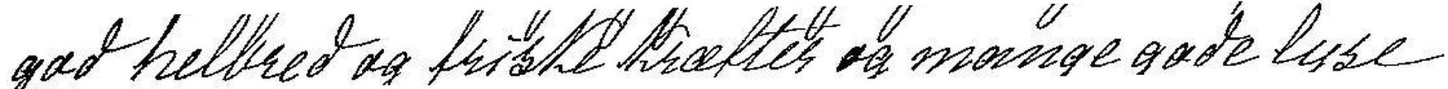god helbred og friske kræfter og mange gode lyse
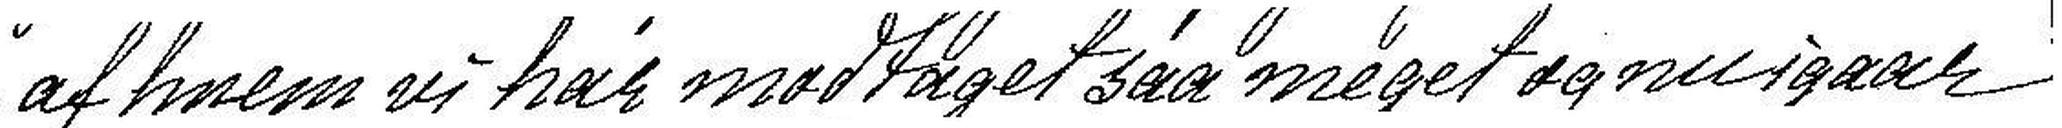af hvem vi har modtaget saa meget og nu igaar
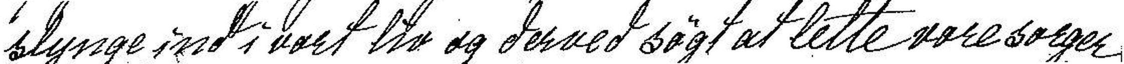slynge ind i vort liv og derved søgt at lette vore sorger
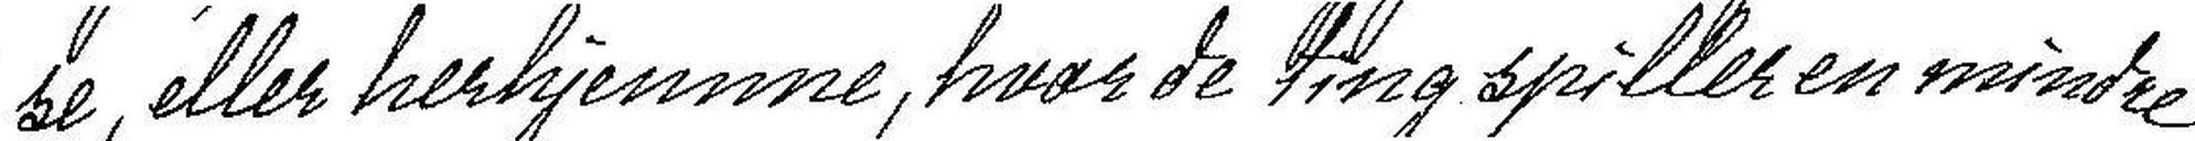se, eller herhjemme, hvor de ting spiller en mindre
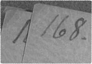1168.
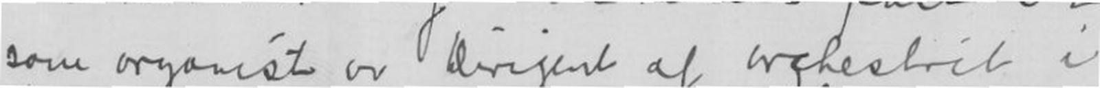som organist og Dirigent af orchestret i
